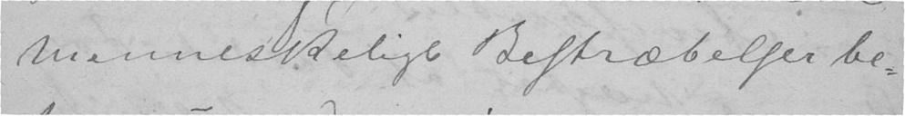menneskelige Bestræbelser be-
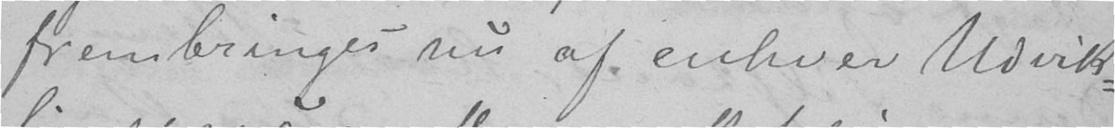frembringes nu af enhver Udvik-
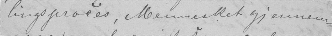lingsproces, Mennesket gjennem-
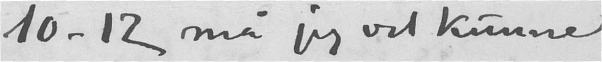10 - 12 må jeg vel kunne
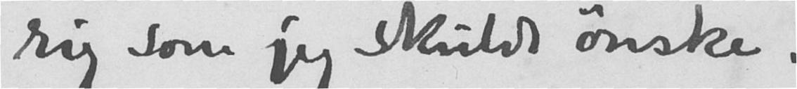rig som jeg skulde ønske.
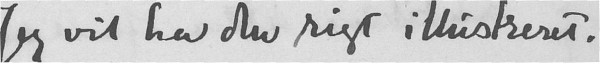Jeg vil ha den rigt illustreret.
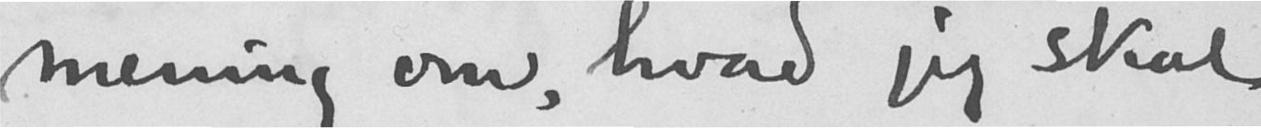mening om hvad jeg skal
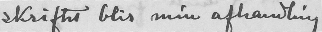skriftet blir min afhandling
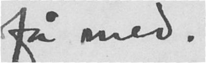få med.
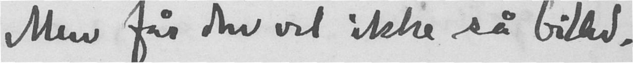Men får den vel ikke så billed-
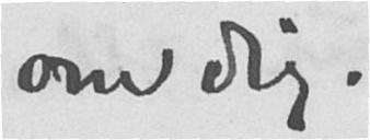om dig.
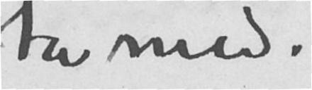ta med.
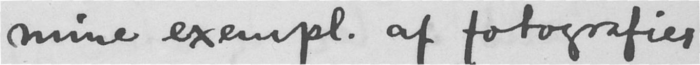mine exempl. af fotografier
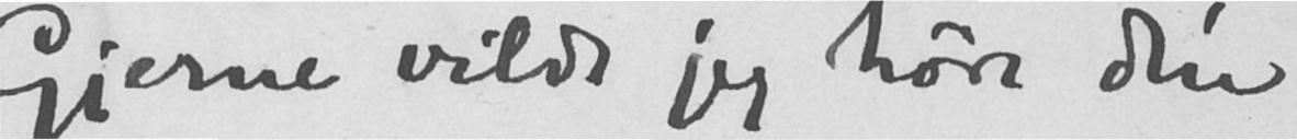Gjerne vilde jeg høre din
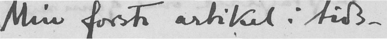Min første artikel i tids-
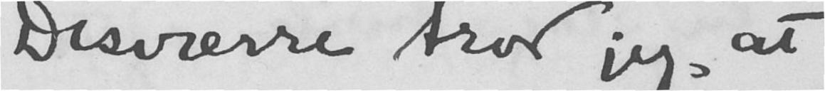Desværre tror jeg, at
